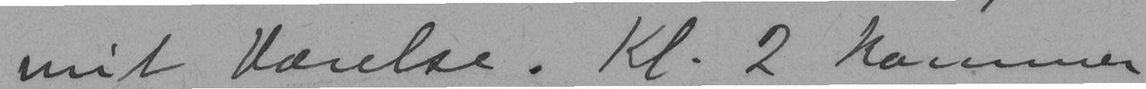mit Værelse. Kl. 2 kommer
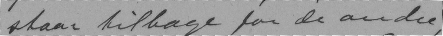staar tilbage for de andre,
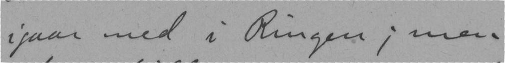igaar med i Ringen; men
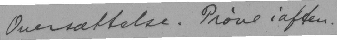Oversættelse. Prøve iaften.
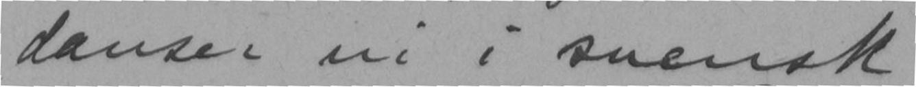danser vi i svensk
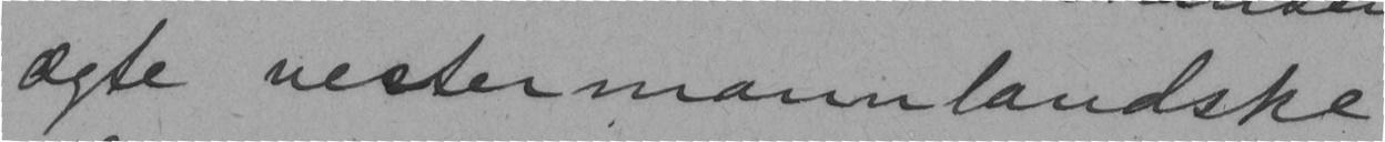ægte vestermannlandske
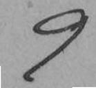9
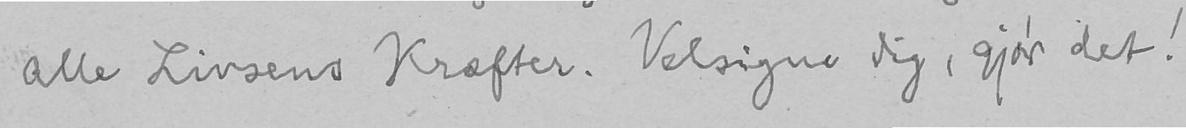alle Livsens Kræfter. Velsigne dig, gjør det!
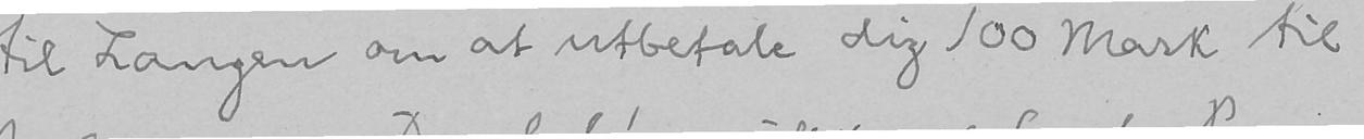til Langen om at utbetale dig 100 Mark til
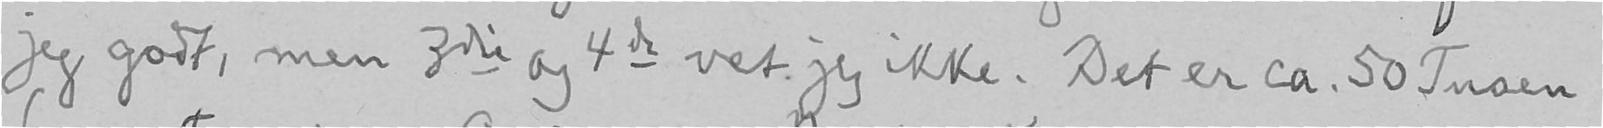jeg godt, men 3die og 4de vet jeg ikke. Det er ca. 50 Tusen
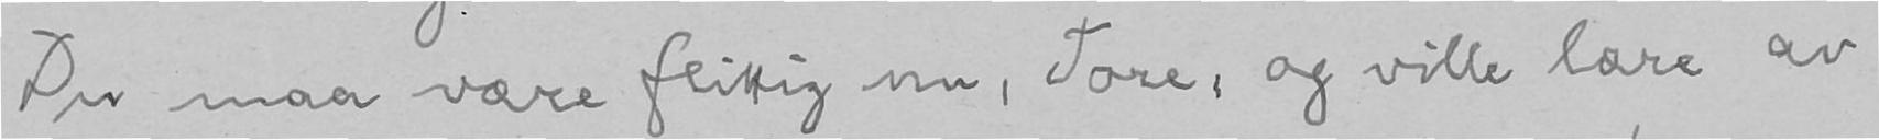Du maa være flittig nu, Tore, og ville lære av
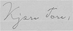Kjære Tore,
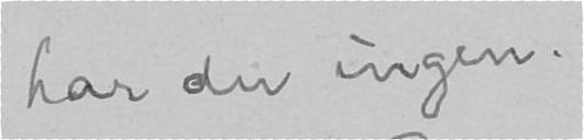har du ingen.
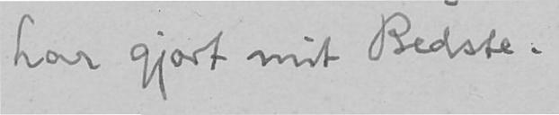har gjort mit Bedste.
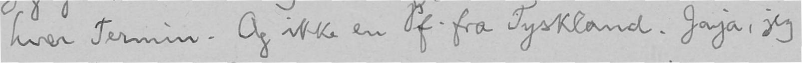hver Termin. Og ikke en Pf. fra Tyskland. Jaja, jeg
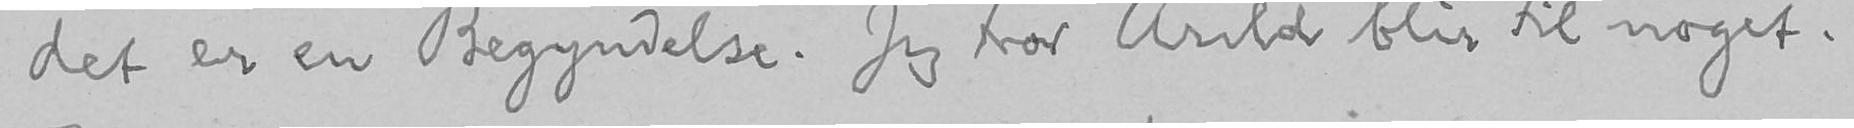det er en Begyndelse. Jeg tror Arild blir til noget.
